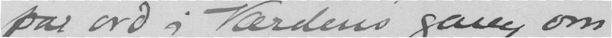par ord i Værdens gang om
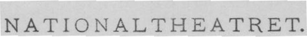NATIONALTHEATRET.
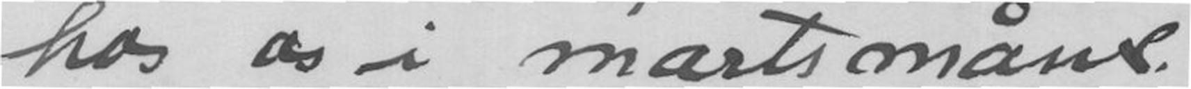hos os i marts måned.
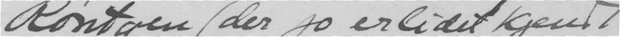Röntgen (der jo er lidet kjendt
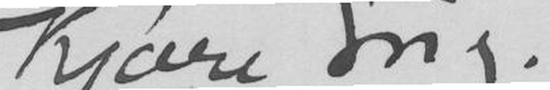Kjære Grieg!
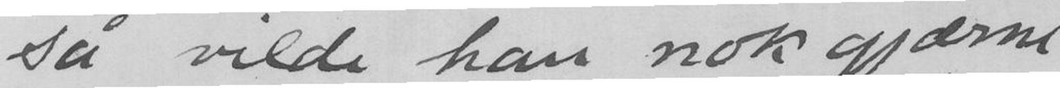så vilde han nok gjærne
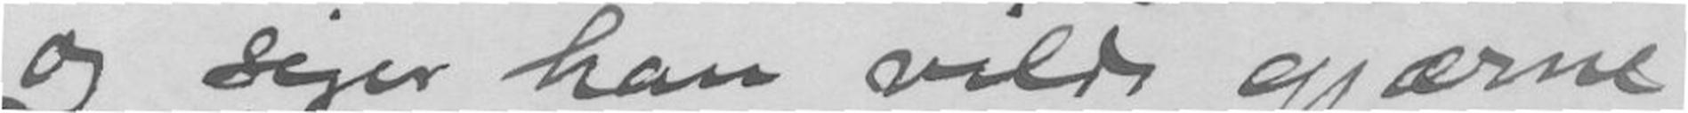og siger han vilde gjærne
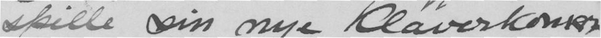spille sin nye Klaverkonsert
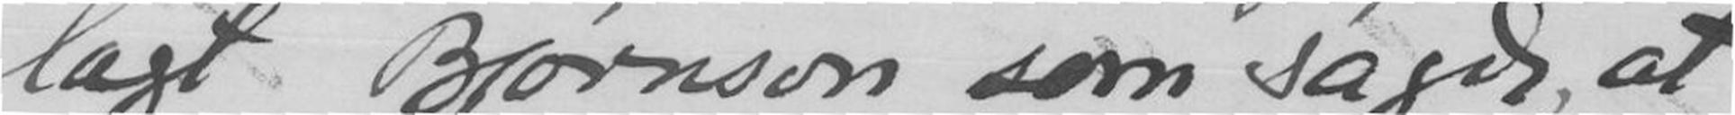lagt Bjørnson som sagde, at
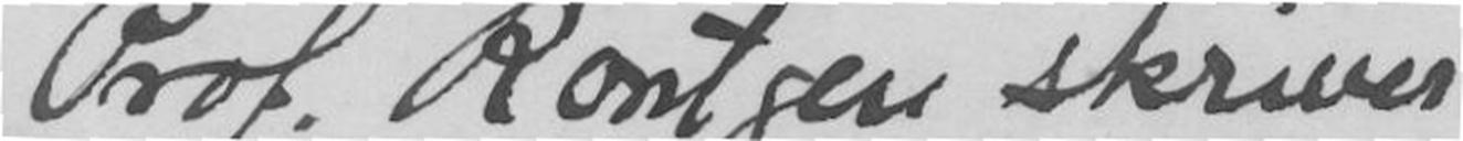Prof. Röntgen skriver
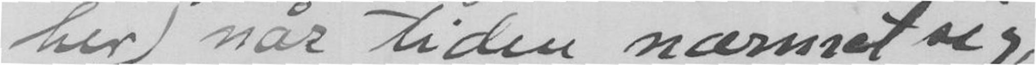her) når tiden nærmet sig,
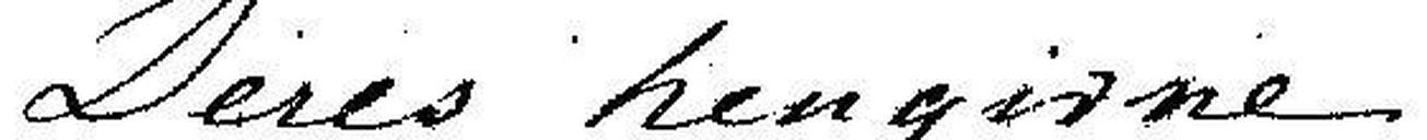Deres hengivne
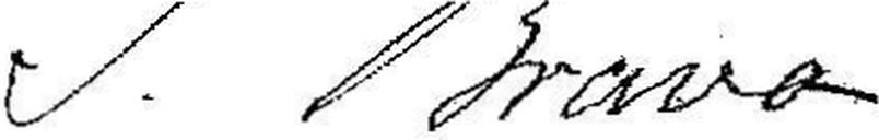J. Bravo
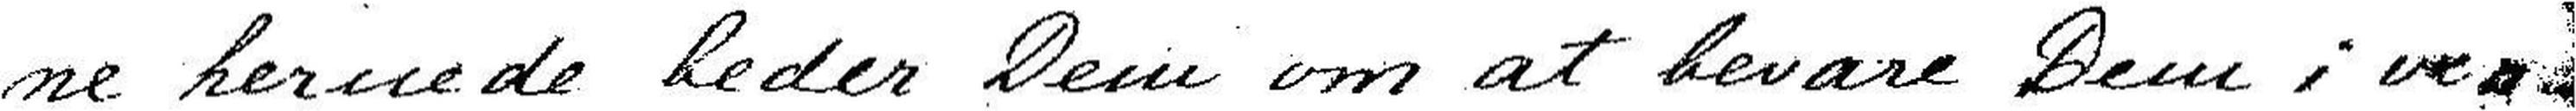ne hernede beder Dem om at bevare Dem i ven-
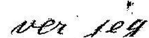ver jeg
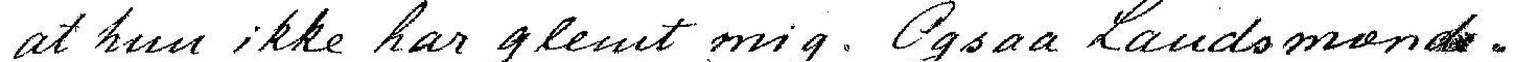at hun ikke har glemt mig. Ogsaa Landsmænde-
